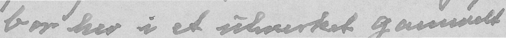bor her i et utmerket gammelt
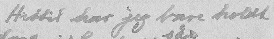Hittil har jeg bare holdt
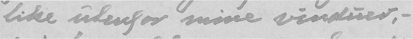like utenfor mine vinduer,-
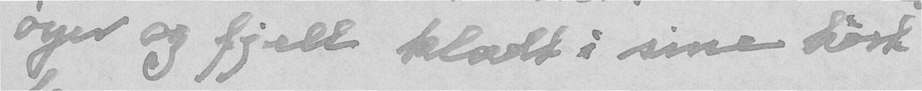øyer og fjell klædt i sine høst-
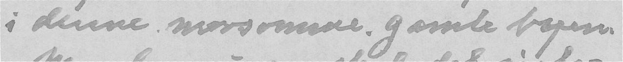i denne morsomme, gamle byen.
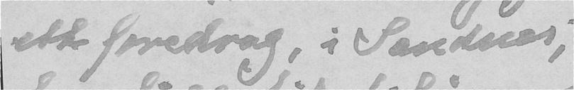ett foredrag, i Sandes
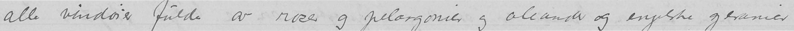alle vinduer fulde av roser og pelargonier og oleander og engelske geranier
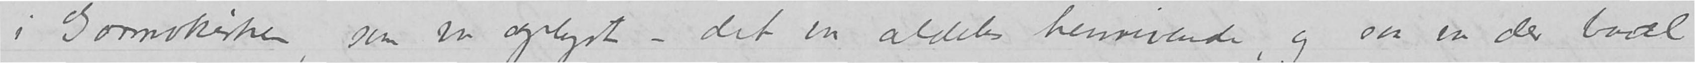i Garmokirken, som var oplyst – det var aldeles henrivende, og saa var der baal
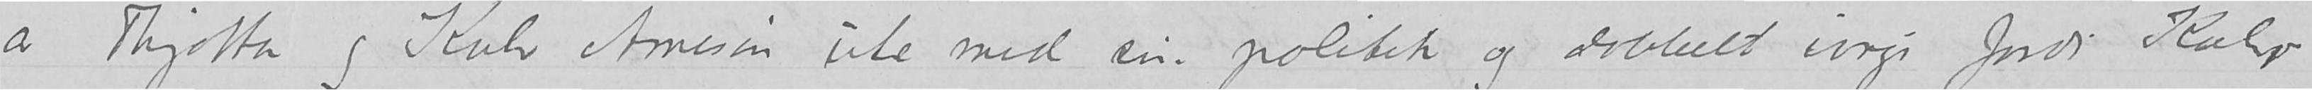av Thjøtta og Kalv Arnesøn ute med sin politik og dobbelt ivrige fordi Kalv
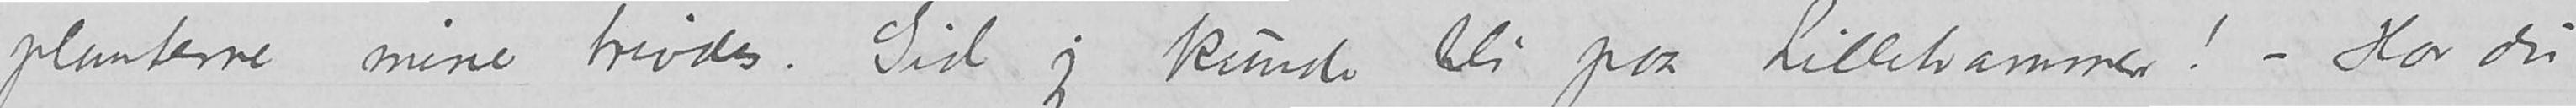planterne mine trivdes.  Gid jeg kunde bli paa Lillehammer! – Har du
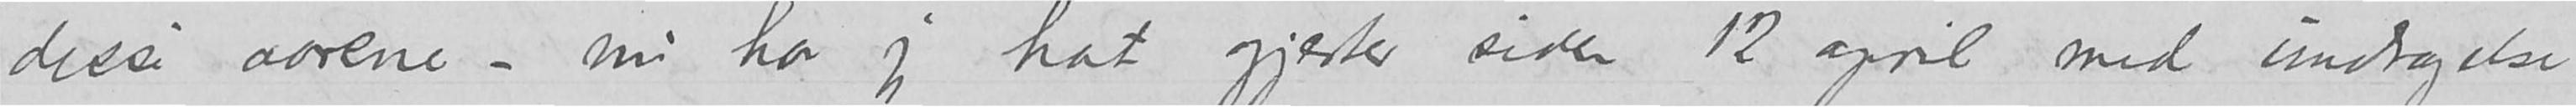disse aarene – nu har jeg hat gjester siden 12. april med undtagelse
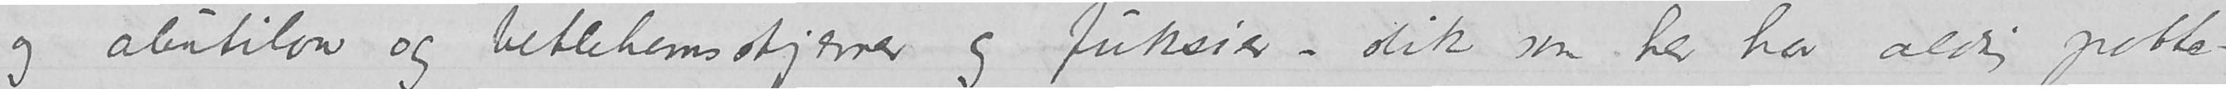og abutilon og betlehemsstjerner og fuksier – slik som her har aldrig potte-
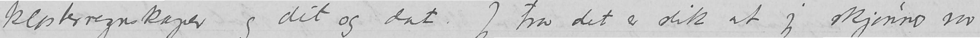klosterregnskaper og dit og dat.  Jeg tror det er slik at jeg skjønner vaar
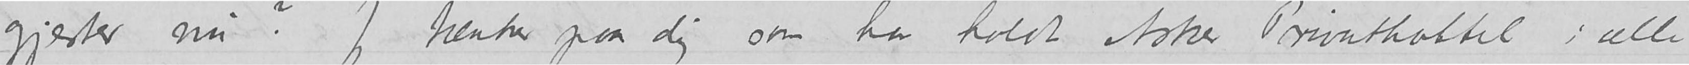gjester nu?  Jeg tænker paa dig som har holdt Asker Privathottel i alle
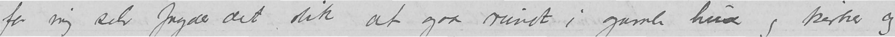for mig selv fryder det slik at gaa rundt i gamle huse og kirker og
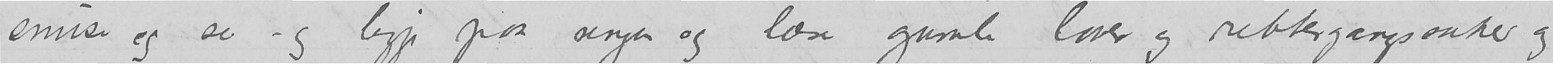snuse og se – og ligge paa sengen og læse gamle lover og rettergangssaker og
